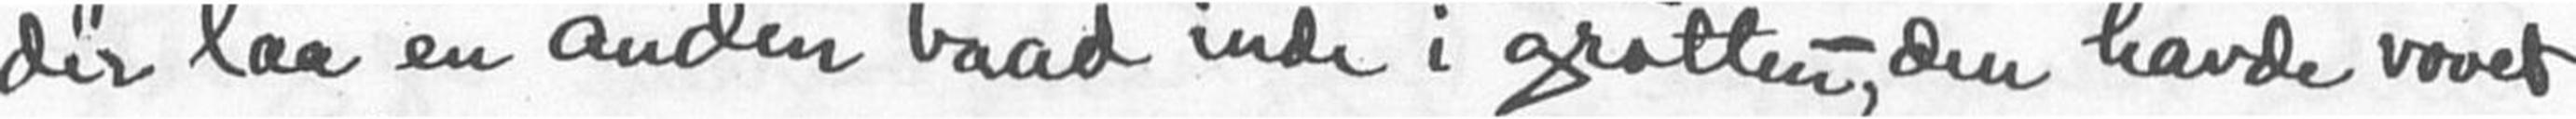der laa en anden baad inde i grotten, - den havde vovet
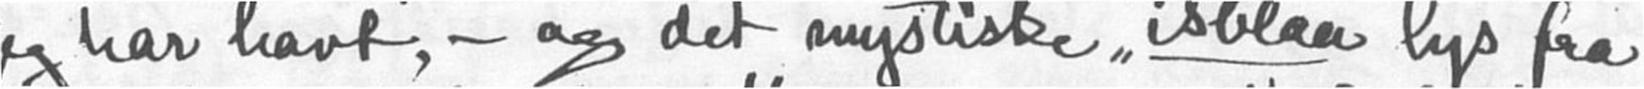jeg har havt, - og det mystiske "isblaa" lys fra
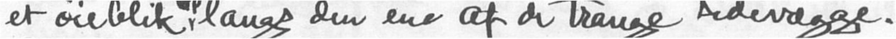et øieblik op lags den ene af de trange sidevægge.
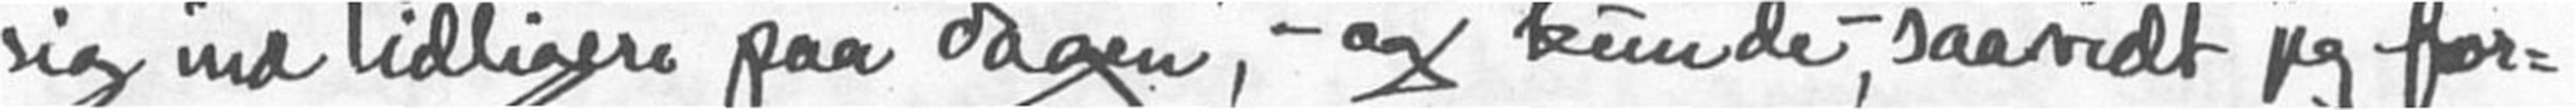sig ind tidligere paa dagen, - og kunde, - saavidt jeg for-
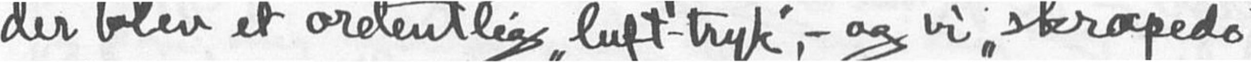der blev et ordentlig "luft-tryk", - og vi "skrapede"
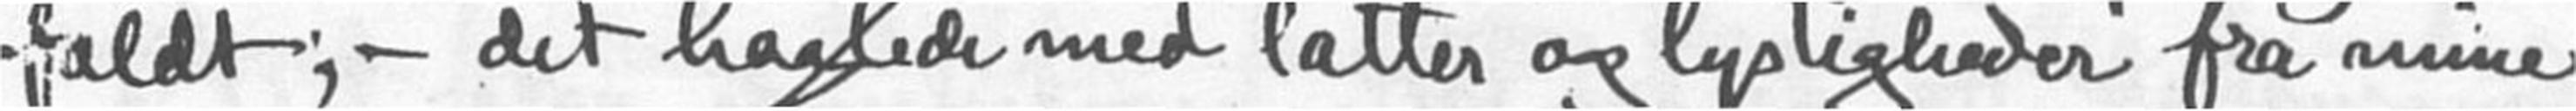faldt; - det haglede med latter og lystigheder fra mine
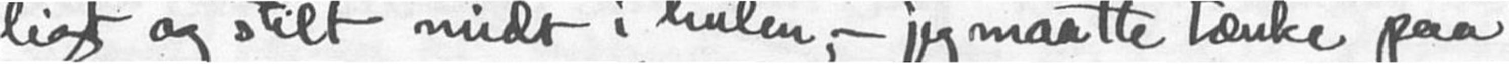ligt og stilt midt i hulen, - jeg maate tænke paa
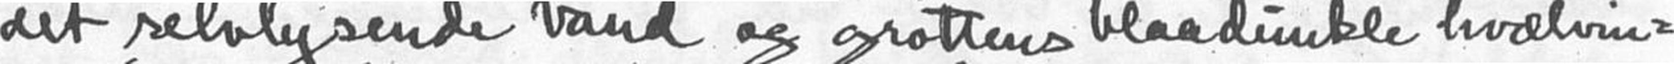det selvlysende vand og grottens blaadunkle hvælvin-
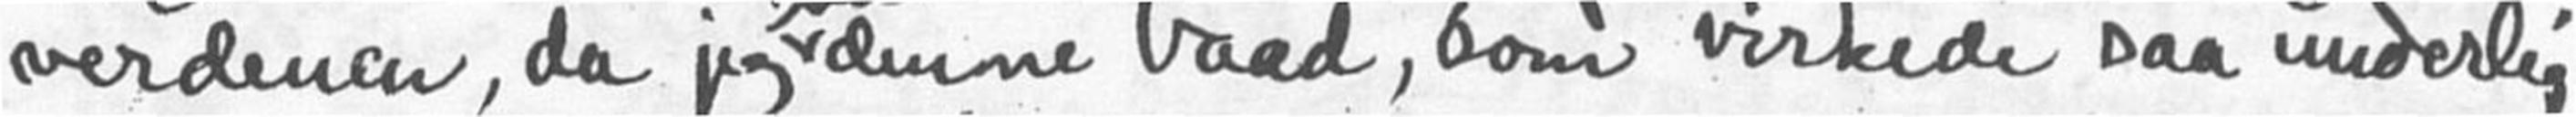verdenen, da jeg saa denne baad, som virkede saa underlig
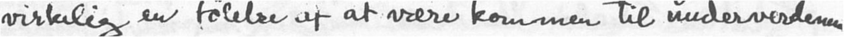virkelig en følelse af at være kommen til underverdenen
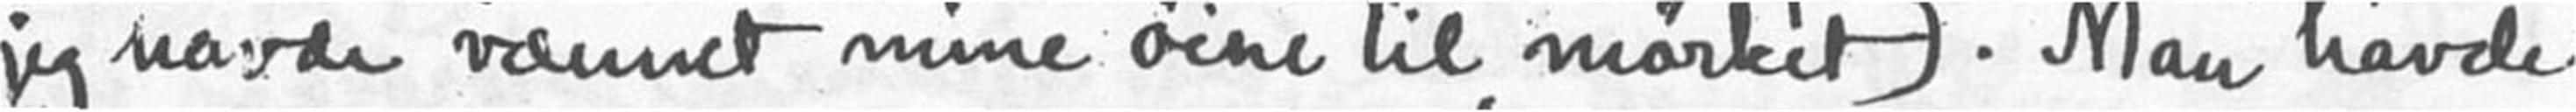jeg havde vænnet mine oine til mørket). Man havde
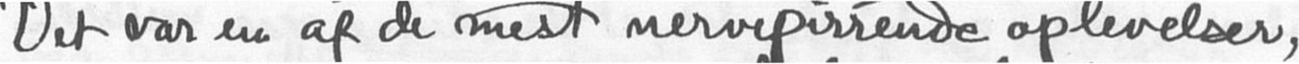Det var en af de mest nervepirrende oplevelser
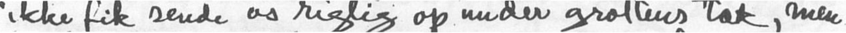ikke fik sende os rigtig op under grottens tak, men
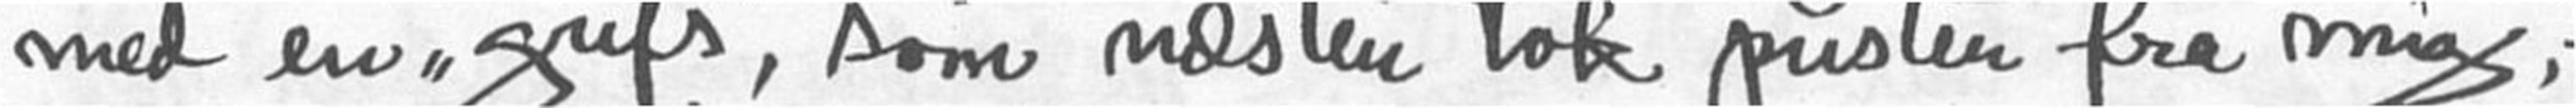med en "gufs", som næsten tok pusten fra mig,-
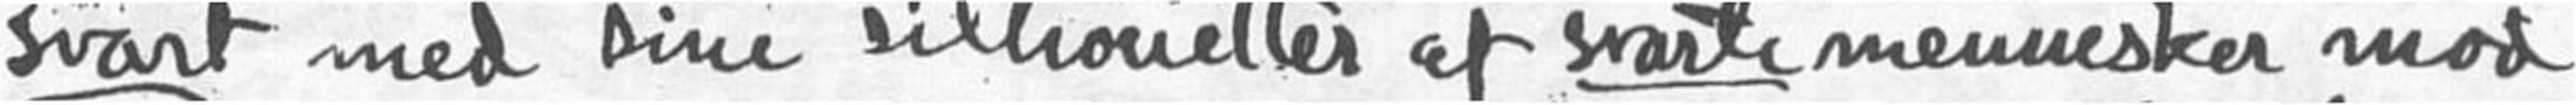svart med sine silhouetter af svarte mennesker mod
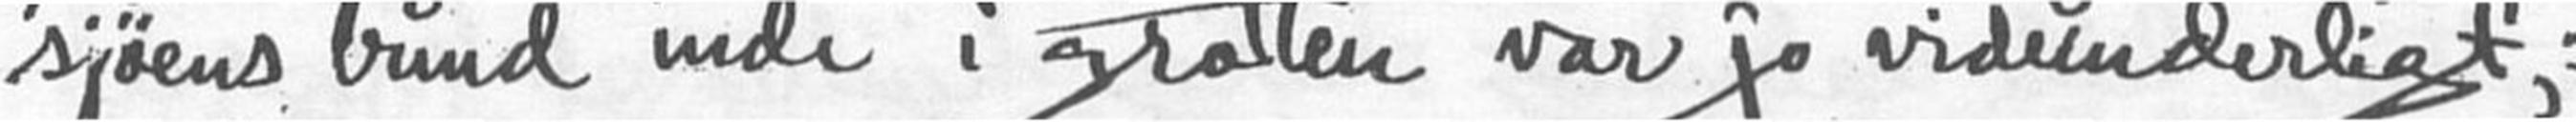sjøens bund inde i grotten var jo vidunderligt; -
